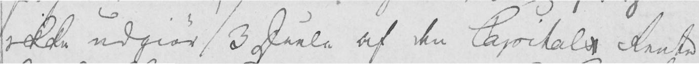ikke udgiør 3 Deele af den Capitals Rente
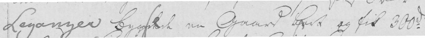Leganger bygslede en Gaard bort og fik 300 rd
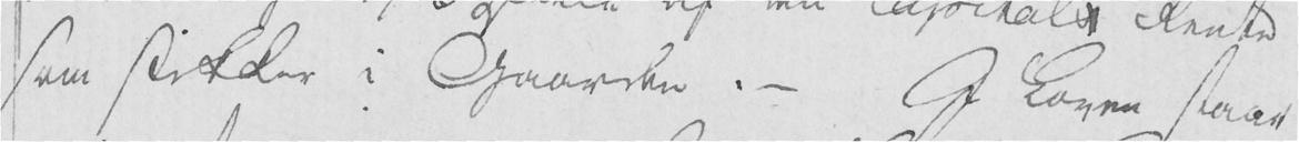som stikker i Gaarden. - I Loven staar
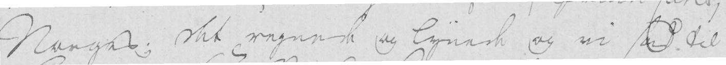Norges; det regnede og lynede og vi sad til
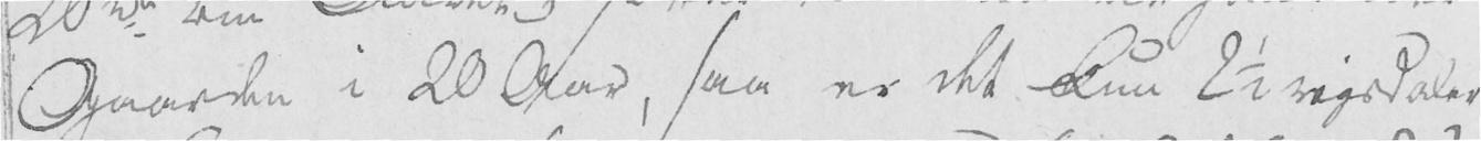Gaarden i 20 Aar, saa er det kun 2 1/2 rigsdaler
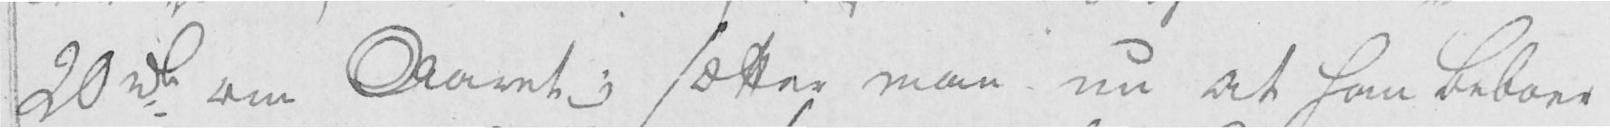20 rde om Aaret; sætter man nu at han beboer
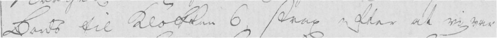Bords til Klokken 6, strax efter at vi var
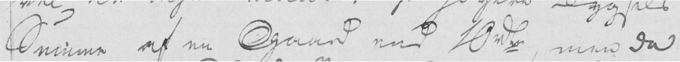Summa af en Gaard end 10 rde, men da
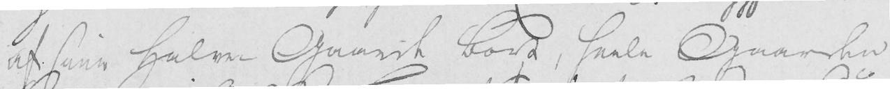af sine halve Gaarde bort, heele Gaarden
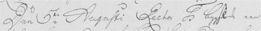Den 5te Augusti Rector Tr. bygslede een
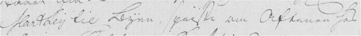Manthey til Byen, spiiste om Aftenen hos
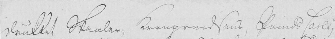drukket Skaaler; Kronprindsens, Prinds Carls,
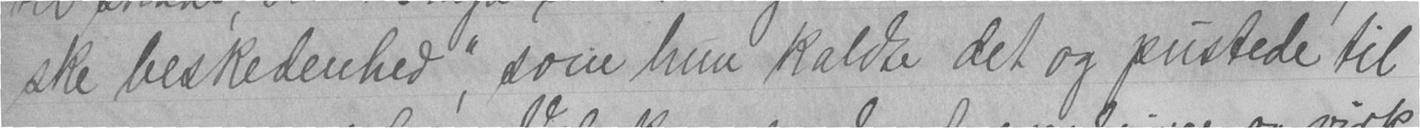ske beskedenhed", som hun kaldte det og pustede til
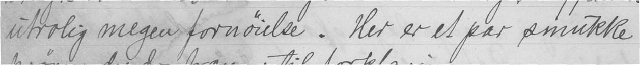utrolig megen fornøielse. Her er et par smukke
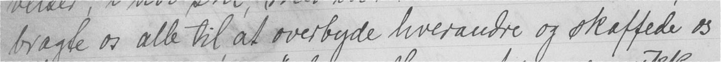bragte os alle til at overbyde hverandre og skaffede os
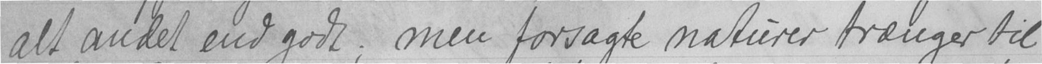alt andet end godt; men forsagte naturer trænger til
